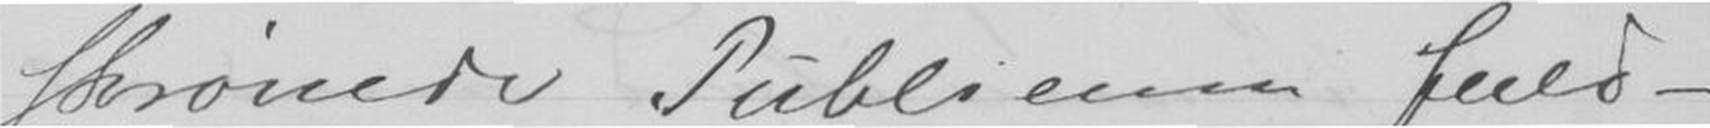skrønede Publicum fuld -
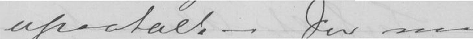upaatalt - Du maa
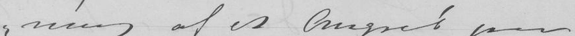ning af et Angreb paa
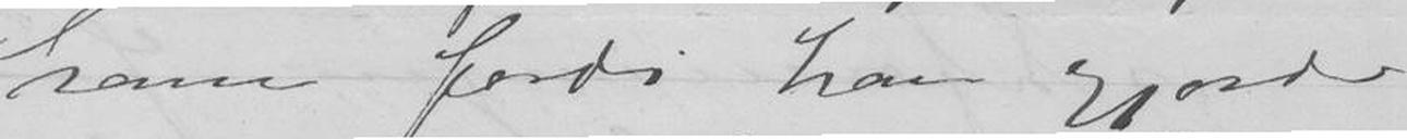ham fordi han gjorde
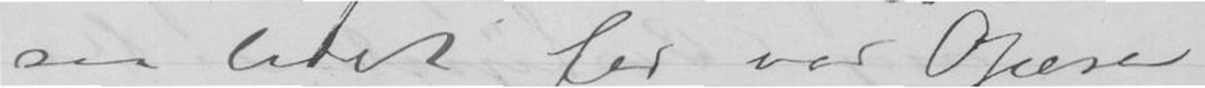saa lidet for vor Opera
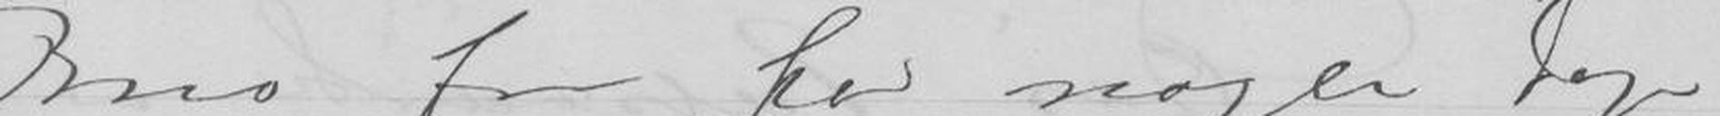Brev fra for nogle Dage
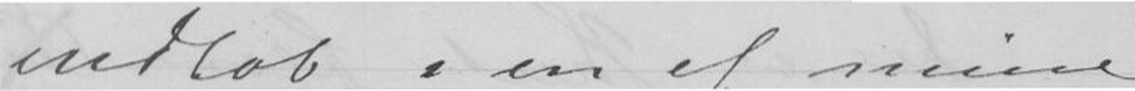indløb i en af mine
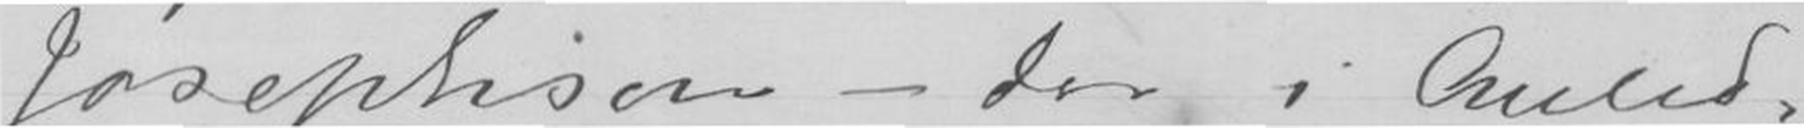Josephson - Der i Anled-
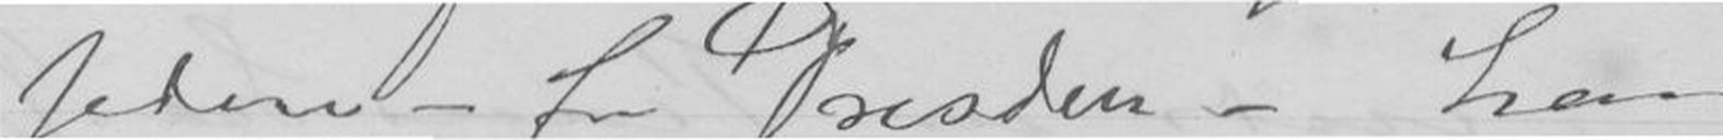siden - fra Dresden - han
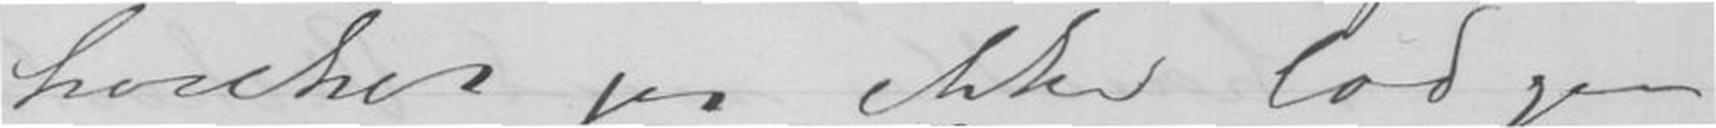hvilket jeg ikke lod gaa
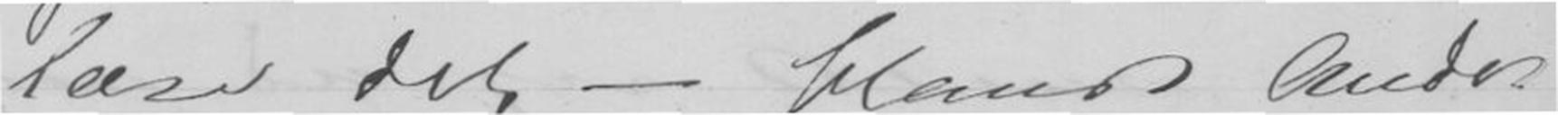læse det - blandt Andet
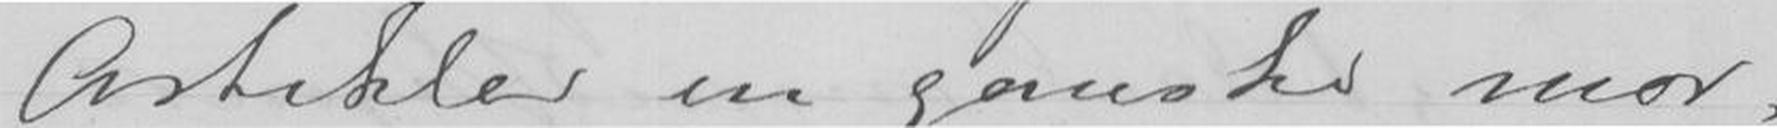Artikler en ganske mor-
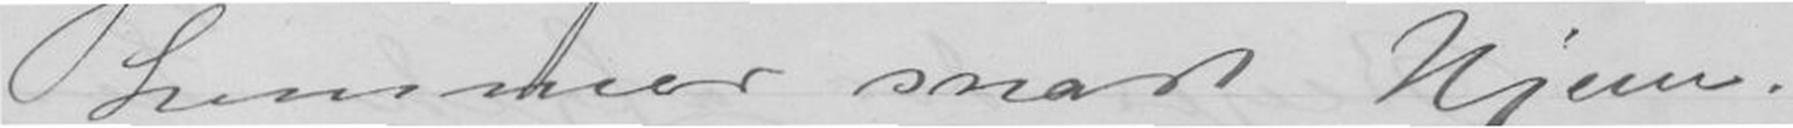kommer snart Hjem.
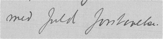med fuld forstaaelse.
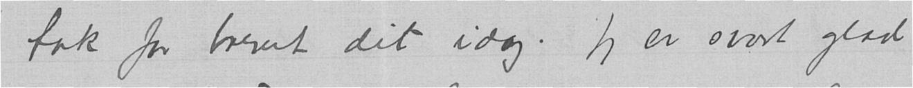tak for brevet dit idag. Jeg er svært glad
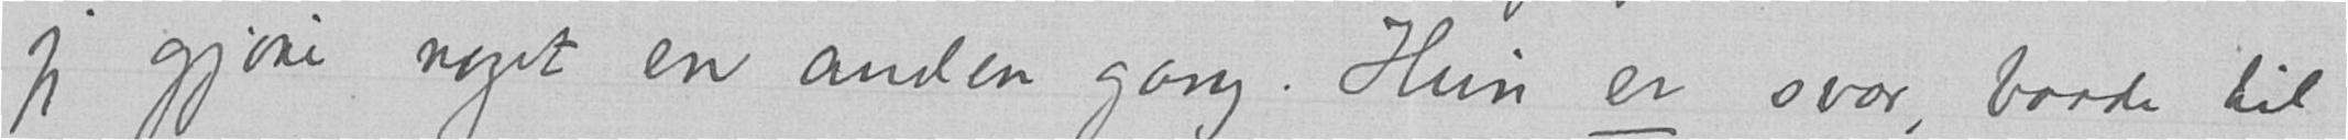jeg gjøre noget en anden gang. Hun er svær, baade til
